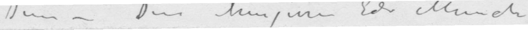Deres – Din hengivne Edv Munch
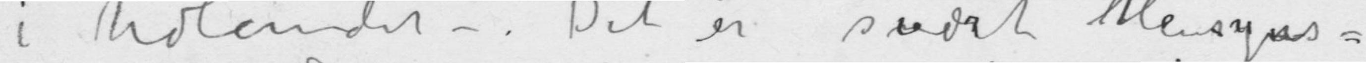i Udlandet – det er svært Hensyns-
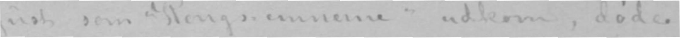Just som «Kongs-emnerne» udkom, døde
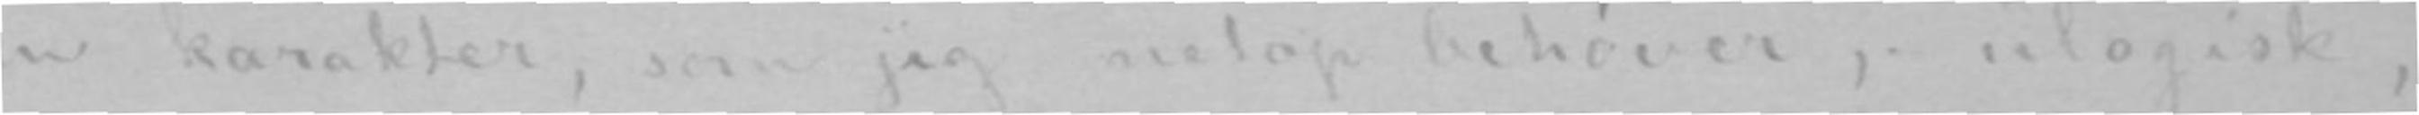en karakter, som jeg netop behøver,- ulogisk,
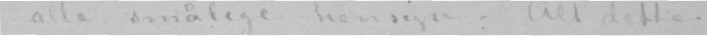had til alle smålige hensyn. Alt dette
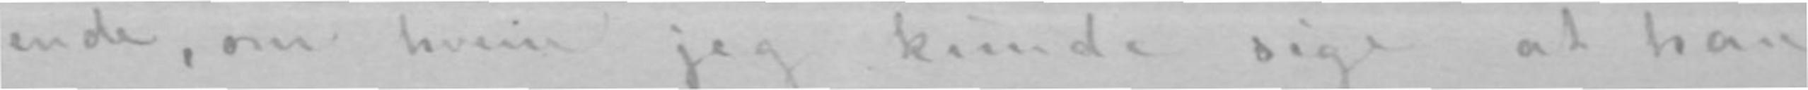ende, om hvem jeg kunde sige at han
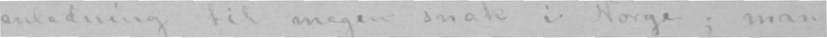anledning til megen snak i Norge; man
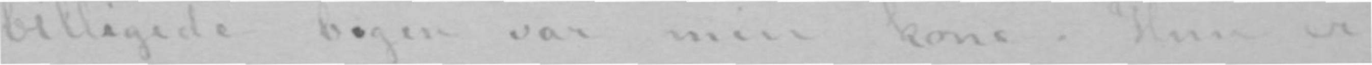billigede bogen var min kone. Hun er
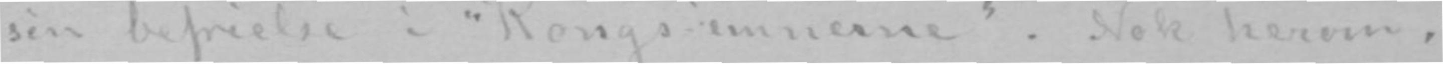sin befrielse i «Kongs-emnerne». Nok herom.
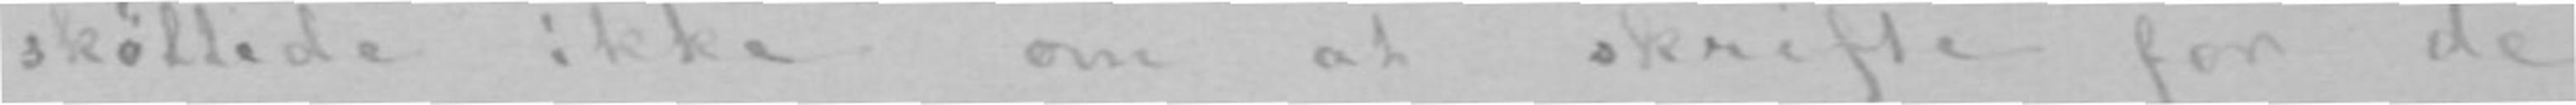skøttede ikke om at skrifte for de
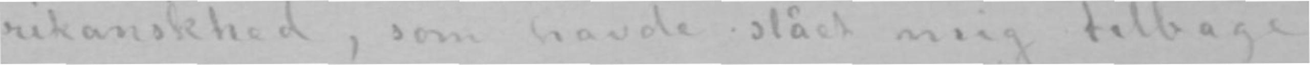rikanskhed, som havde slået mig tilbage
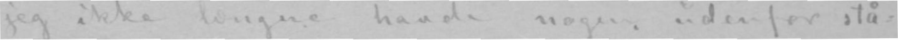jeg ikke længere havde nogen udenfor stå-
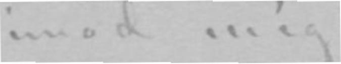imod mig.
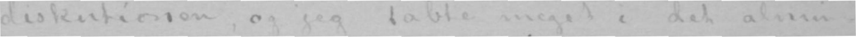diskutionen, og jeg tabte meget i det almin-
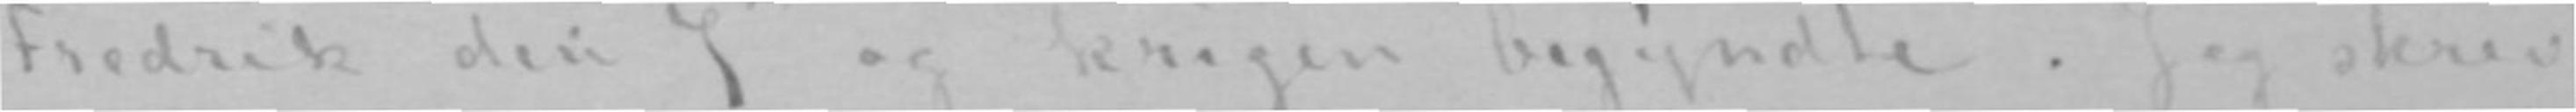Fredrik den 7de og krigen begyndte. Jeg skrev
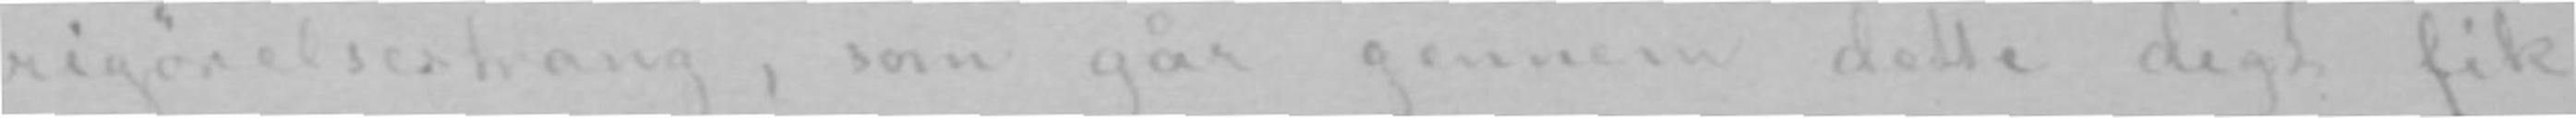frigørelsestrang, som går gennem dette digt fik
